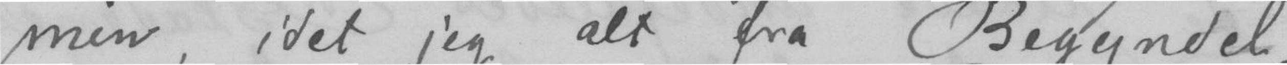min, idet jeg alt fra Begyndel-
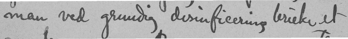man ved grundig desinficering bruke et
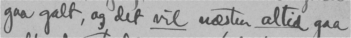gaa galt, og det vil næsten altid gaa
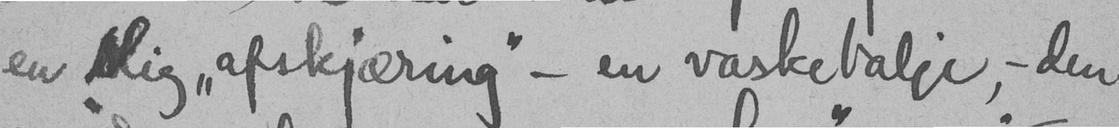en slig "afskjæring" - en vaskebalje, - den
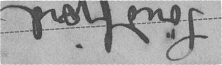Søndfjord
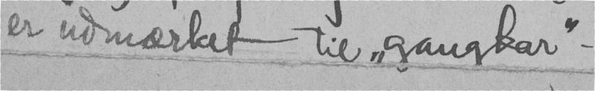er udmærket til "gangkar" -
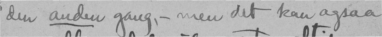den anden gang, - men det kan ogsaa
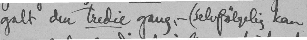galt den tredie gang, - (selvfølgelig kan
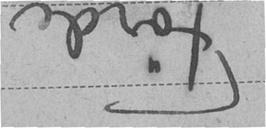Førde
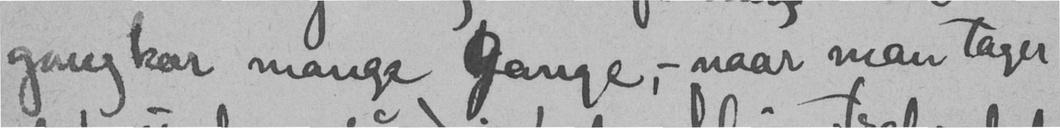gangkar mange gange, - naar man tager
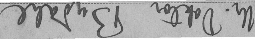Hr. Doktor Bydahl
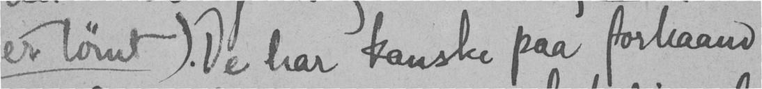er tømt). De har kanske paa forhaand
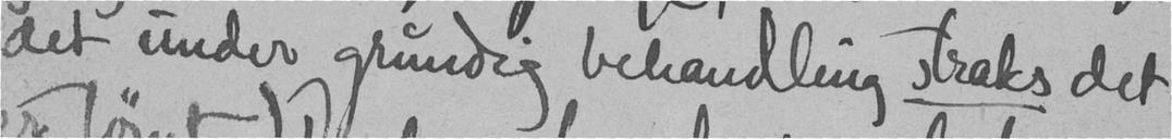det under grundig behandling straks det
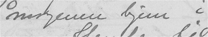morgenen hjem i
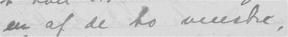en af de to venstre,
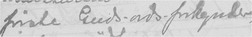første Guds-ords-forkynder,
